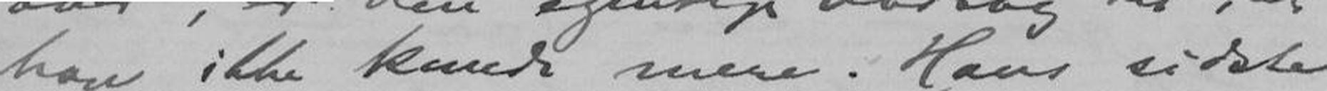han ikke kunde mere. Hans sidste
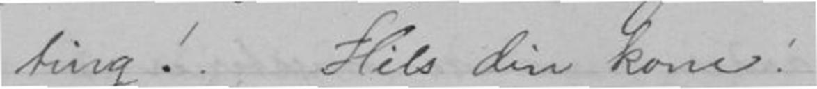ting! Hils din kone!
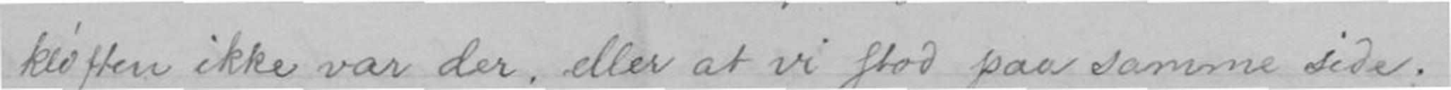kløften ikke var der eller at vi stod paa samme side:
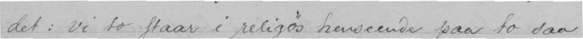det: Vi to staar i religøs henseende paa to saa
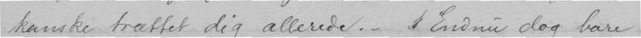kanske trættet dig allerede. - Endnu dog bare
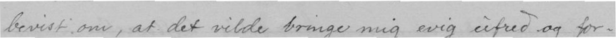bevist om, at det vilde bringe mig evig ufred og for-
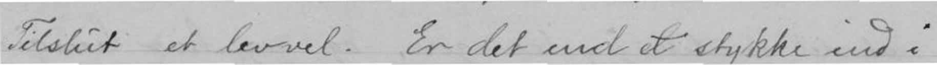Tilslut et levvel. Er det end et stykke ind i
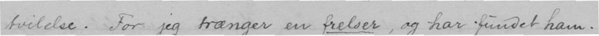tvilelse. For jeg trænger en frelser, og har fundet ham.
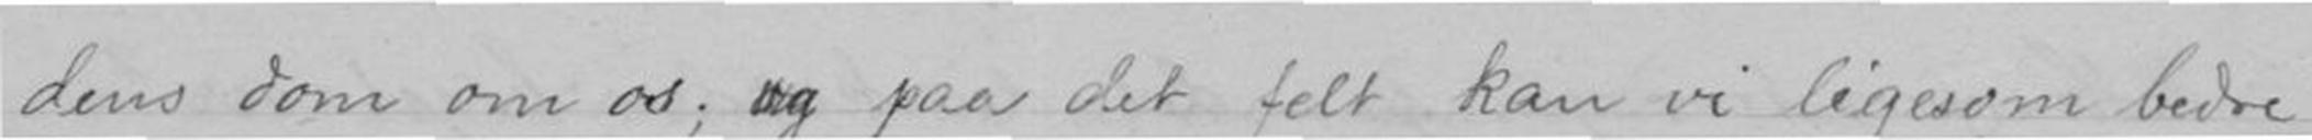dens dom om os; og paa det felt kan vi ligesom bedre
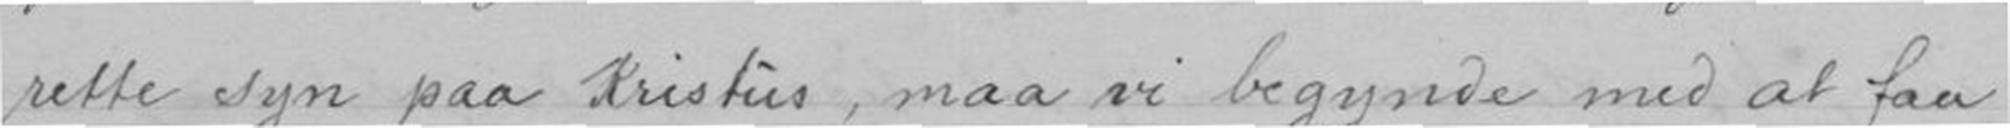rette syn paa Kristus, maa vi begynde med at faa
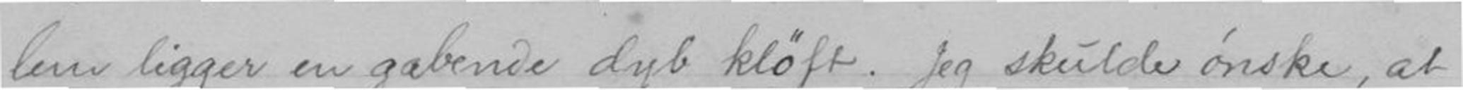mig os som en gabende dyb kløft. Jeg skulde ønske, at
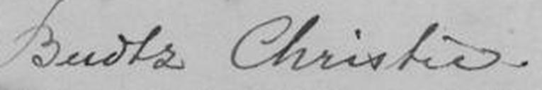Budtz Christie
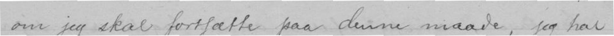om jeg skal fortsætte paa denne maade, jeg har
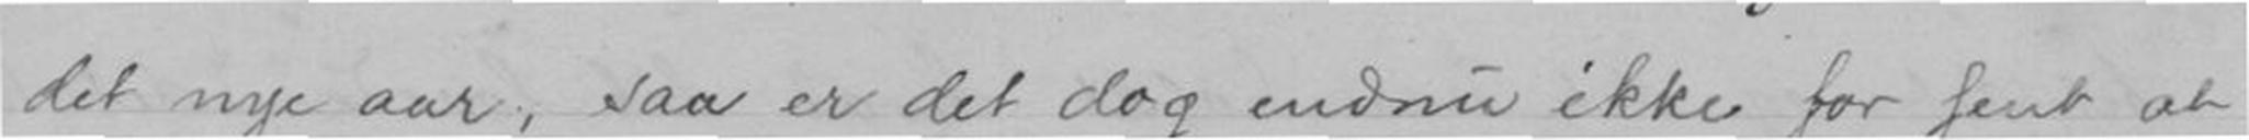det nye aar, saa er det dog endnu ikke for sent at
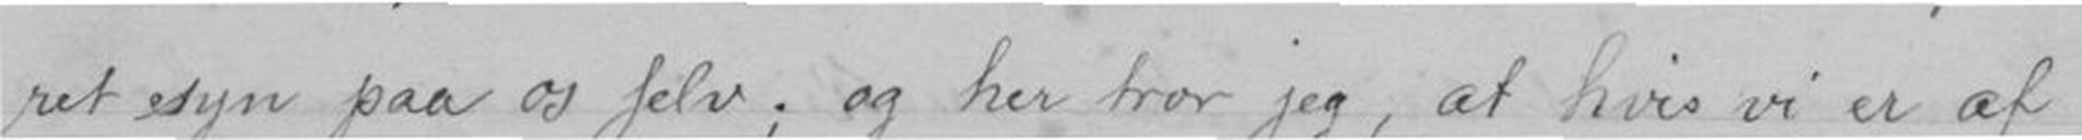ret syn paa os selv; og her tror jeg, at hvis vi er af
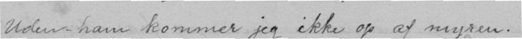Uden ham kommer jeg ikke op af myren.
